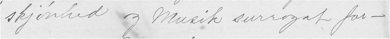skjønhed og Musik surrogat for —
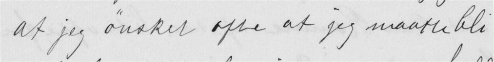at jeg ønsker ofte at jeg maatte bli
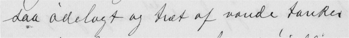saa ødelagt og træt af vonde tanker
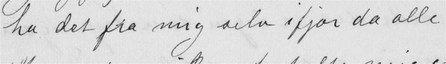ha det fra mig selv ifjor da alle
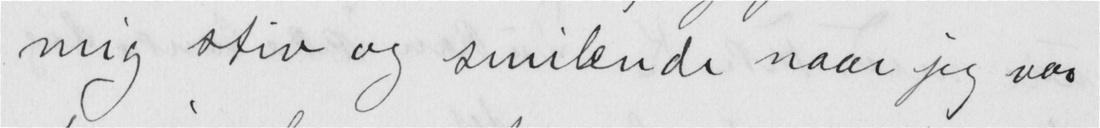mig stiv og smilende naar jeg var
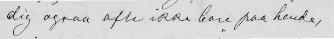dig ogsaa ofte ikke bare paa hende,
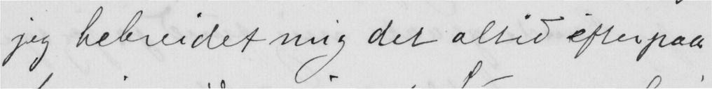jeg bebreidet mig det altid efterpaa
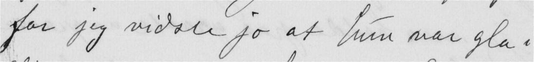for jeg vidste jo at hun var gla i
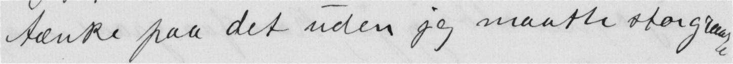tænke paa det uden jeg maatte storgraate
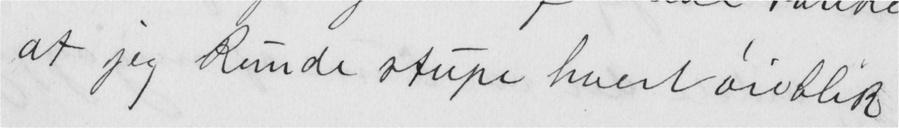at jeg Kunde stupe hvert øieblik
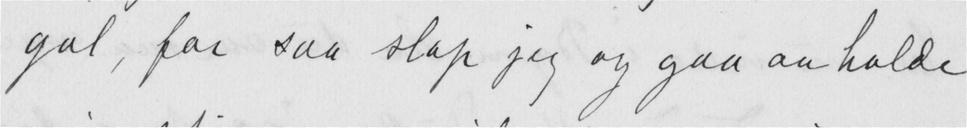gal, for saa slap jeg og gaa anholde
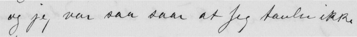og jeg var saa saar at Jeg taulie ikke
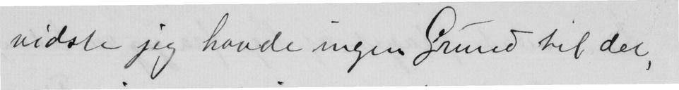vidste jeg havde ingen Grund til det,
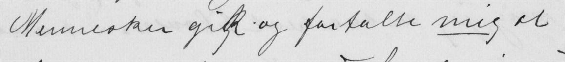Mennesker gik og fortalte mig at
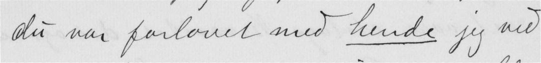de var forlovet med hende jeg ved
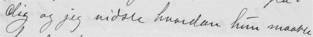dig og jeg vidste hvordan hun maatte
